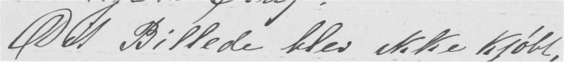Dit Billede blev ikke kjøbt,
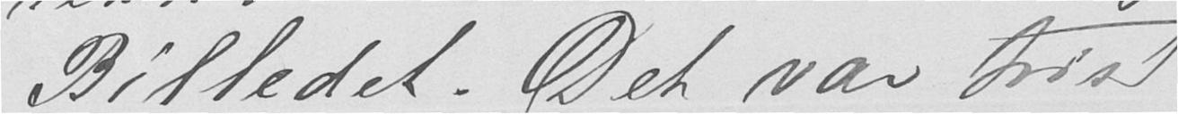Billedet. Det var trist,
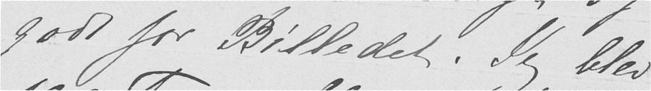godt for Billedet. Jeg blev
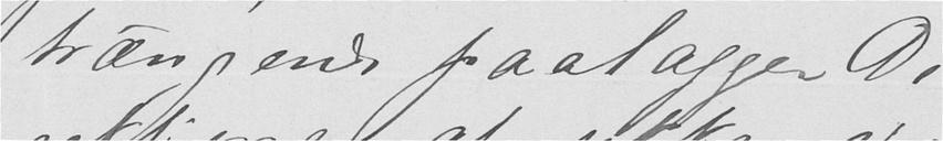trængende paalægger Di-
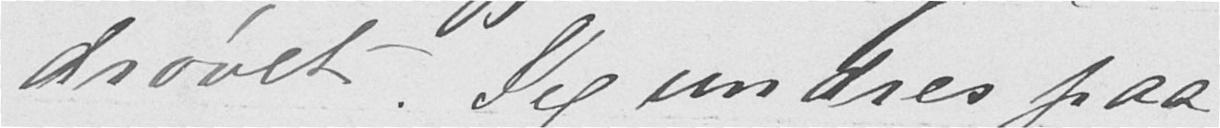drøvet. Jeg undres paa,
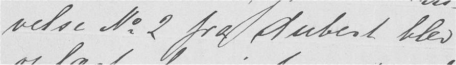velse No 2 fra Aubert blev
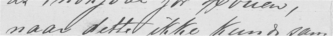naar dette ikke kunde sam-
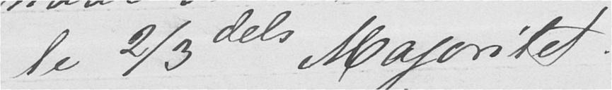le 2/3dels Majoritet.
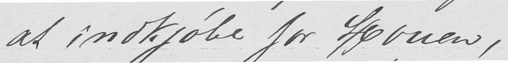at indkjøbe for Houen,
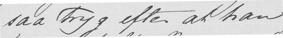saa tryg efter at han
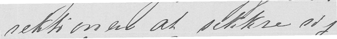rektionen at sikkre sig
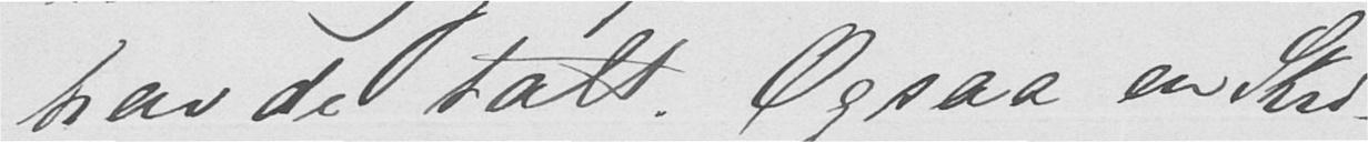havde talt. Ogsaa en Skri-
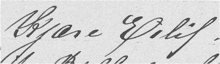Kjære Eilif.
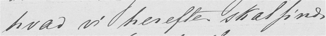hvad vi heretter skal finde
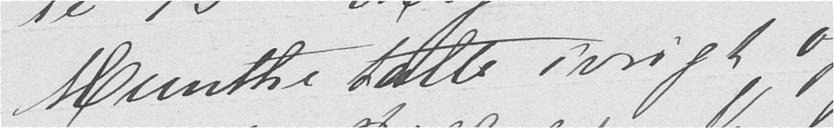Munthe talte ivrigt og
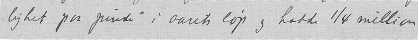lighet paa pinde" i aarets løp og hadde 1/4 million
